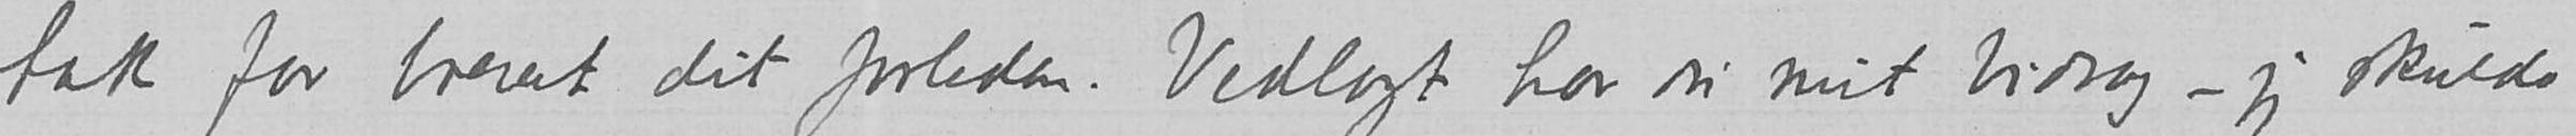tak for brevet dit forleden. Vedlagt har du mit bidrag – jeg skulde
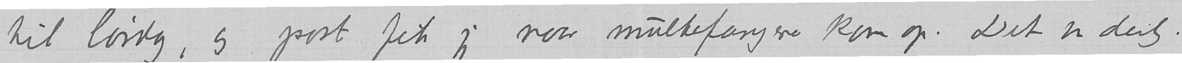til lørdag, og post fik jeg naar multefangere kom op. Det var deilig!
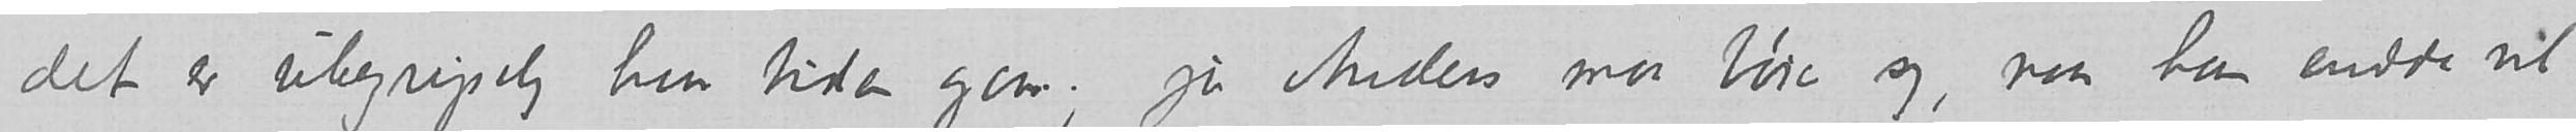– det er ubegripelig hvor tiden gaar; ja Anders maa bøie sig, naar han endda vil
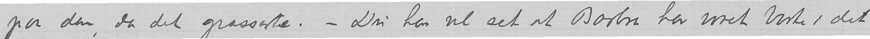paa dem, da det grasserte. – Du har vel set at Barbra har været borte i det
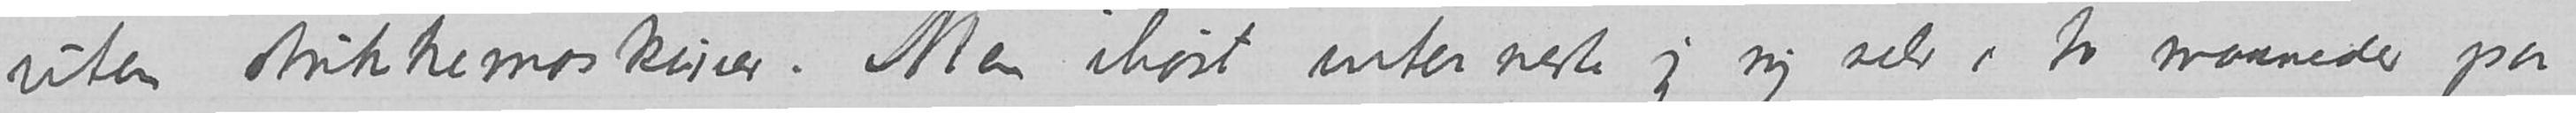uten strikkemaskiner. Men ihøst internerte jeg mig selv i to maaneder paa
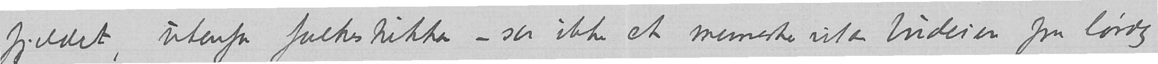fjeldet, utenfor folkskikken - saa ikke et menneske uten budeien fra lørdag
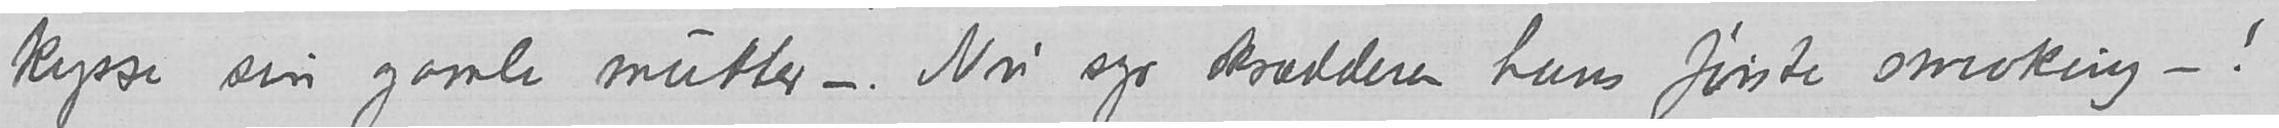kysse sin gamle mutter –. Nu syr skrædderen hans første smoking –!
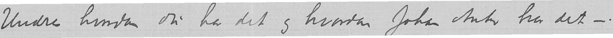Undres hvordan du har det og hvordan Johan Anker har det –.
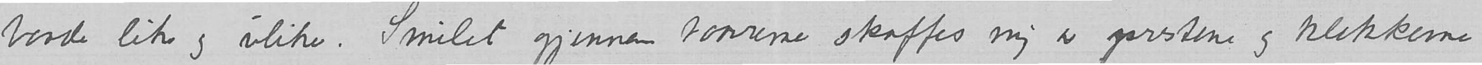baade like og ulike. Smilet gjennem taarerne skaffes mig av prestene og klokkerne
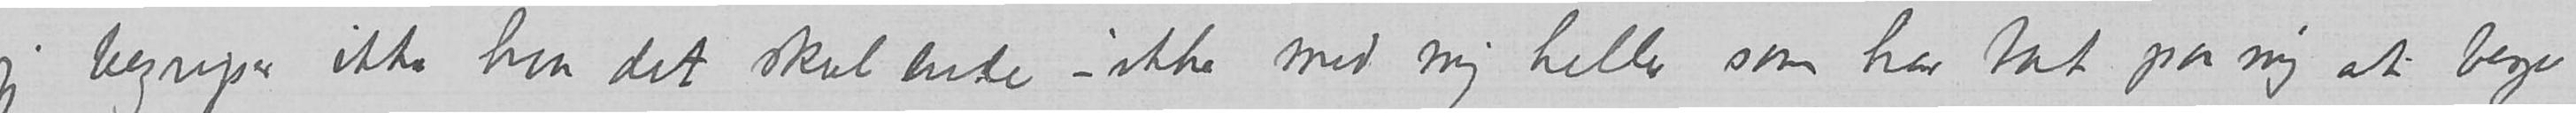jeg begriper ikke hvor det skal ende – ikke med mig heller som har tat paa mig at berge
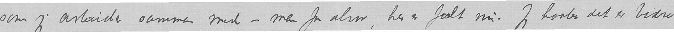som jeg arbeider sammen med – men for alvor, her er fælt nu. Jeg haaber det er bedre
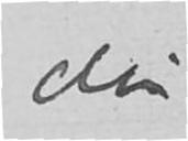din
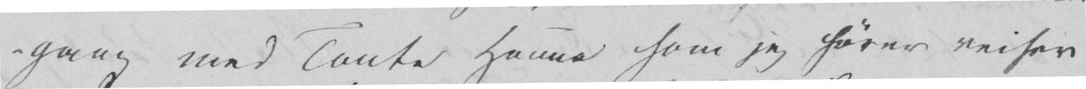gang med Tante Hanne som jeg hører reiser
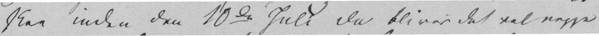skee inden den 10de Juli da bliver det vel neppe
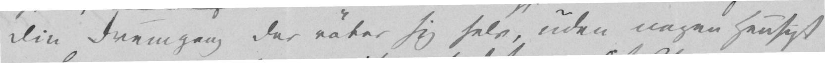Din Fremgang der røber sig selv, uden nogen Hensigt
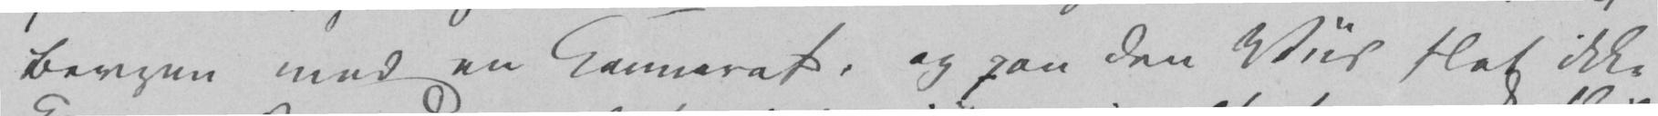Bergen med en Kamerat, og paa den Viis slet ikke
